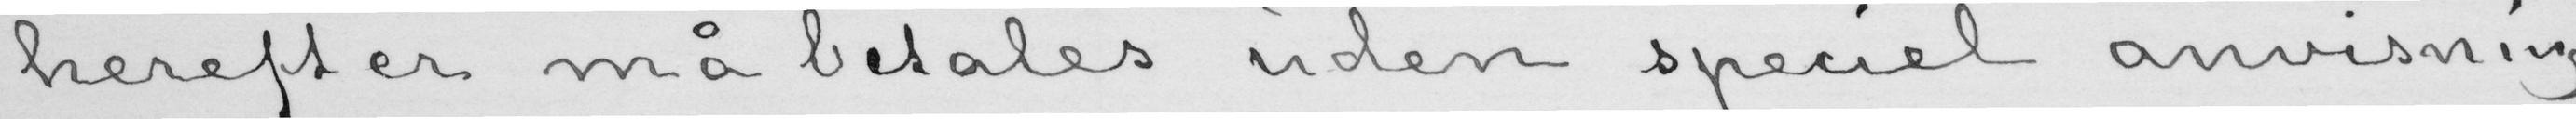herefter må betales uden speciel anvisning
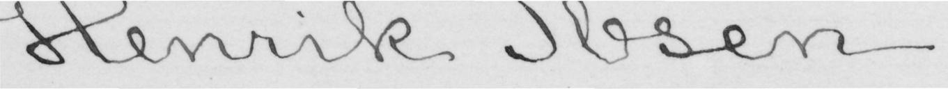Henrik Ibsen.
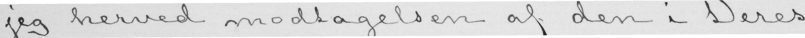jeg herved modtagelsen af den i Deres
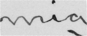mig
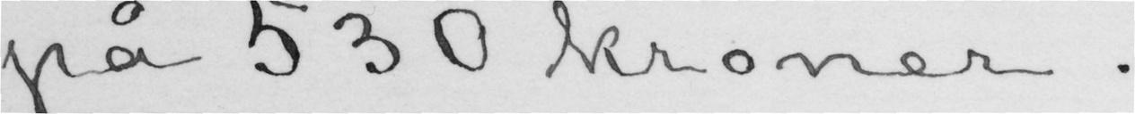på 530 kroner.
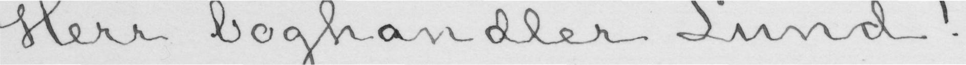Herr boghandler Lund!
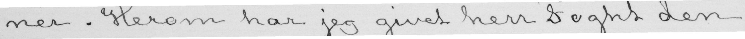ner. Herom har jeg givet herr Foght den
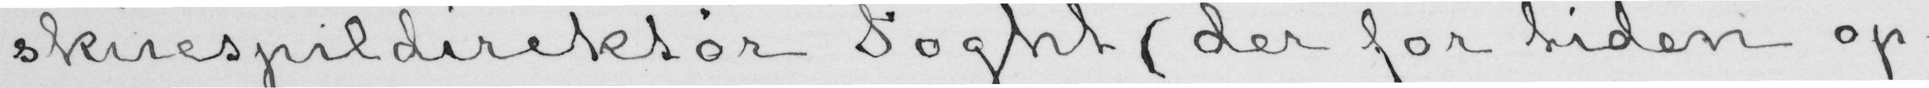skuespildirektør Foght (der for tiden op-
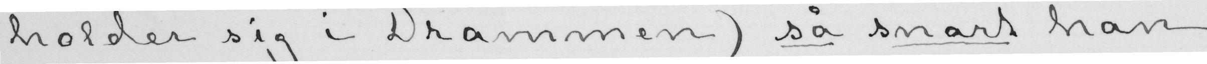holder sig i Drammen) så snart han
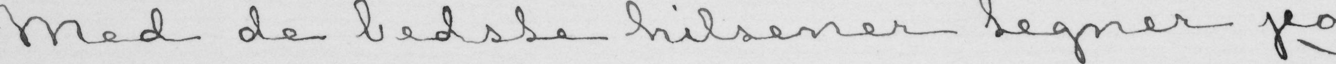Med de bedste hilsener tegner jeg
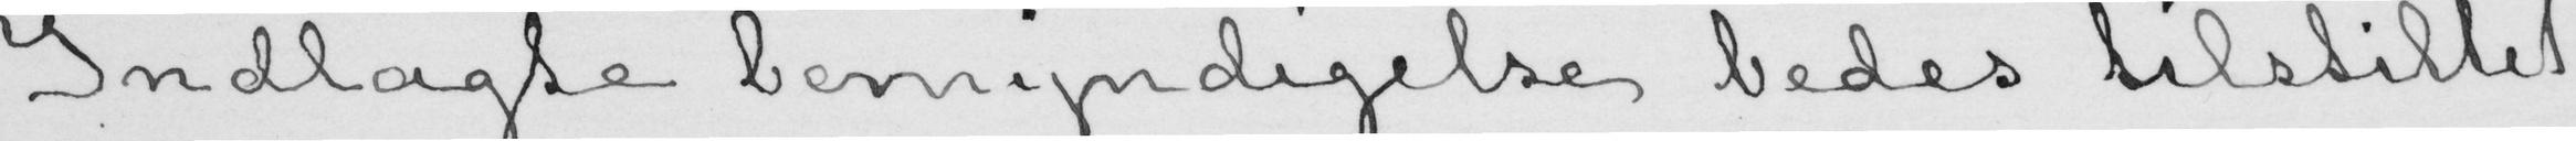Indlagte bemyndigelse bedes tilstillet
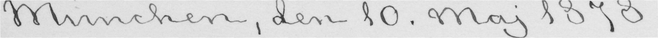München, den 10. Maj 1878.
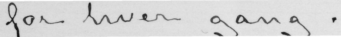for hver gang.
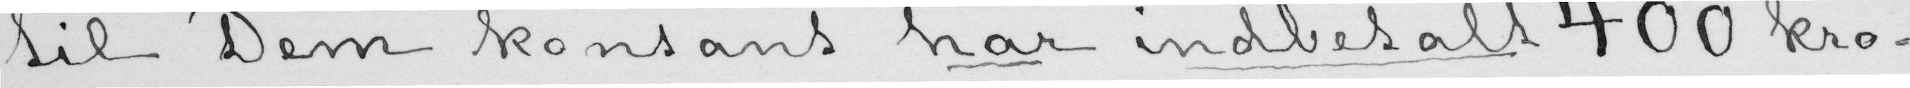til Dem kontant har indbetalt 400 kro-
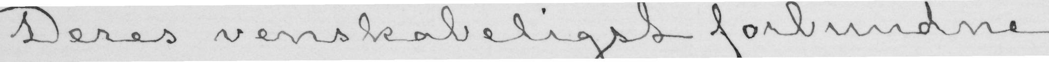Deres venskabeligst forbundne
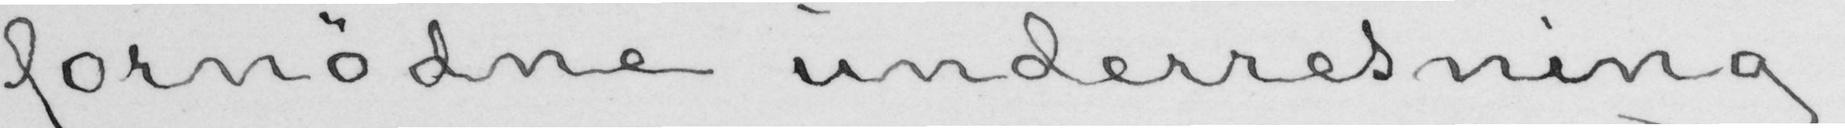fornødne underretning.
